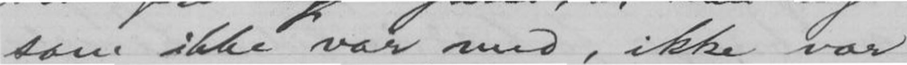som ikke var med, ikke var
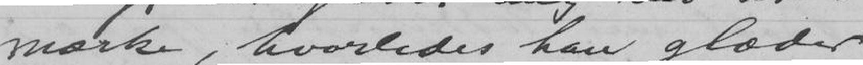mærke, hvorledes han glæder
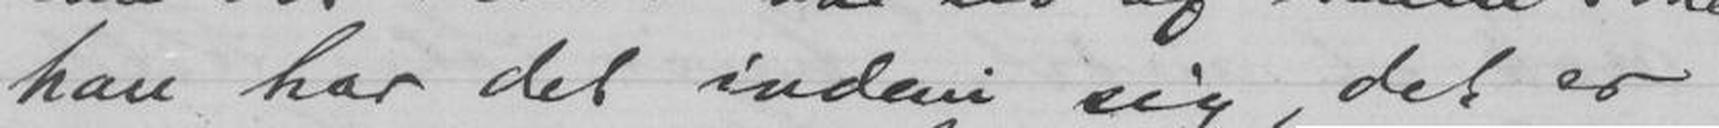han har det indeni sig, det er
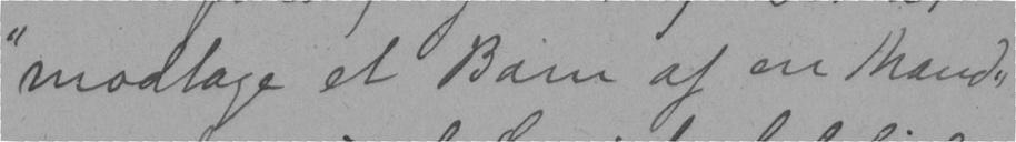"modtage et Barn af en Mand
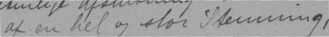af en hel og stor Stemning,
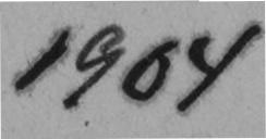1904
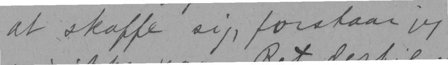at skaffe sig, forstaar jeg
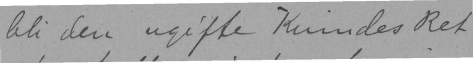bli den ugifte Kvindes Ret
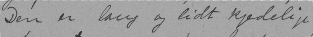Den er lang og lidt kjedeligt
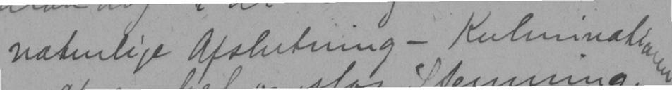naturlige Afslutning - Kulminationen
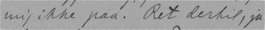mig ikke paa. Ret dertil, ja
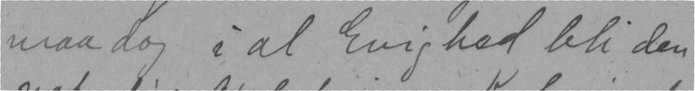maa dog i al Evighed bli den
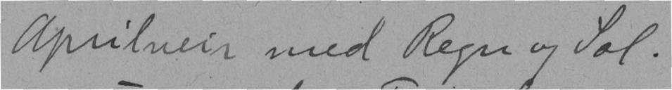Aprilveir med Regn og Sol.
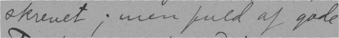skrevet; men fuld af gode
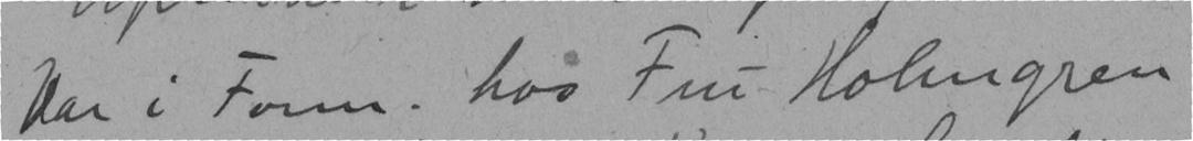Var i Form. hos Fru Holmgren
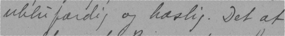ublufærdig og hæslig. Det at
